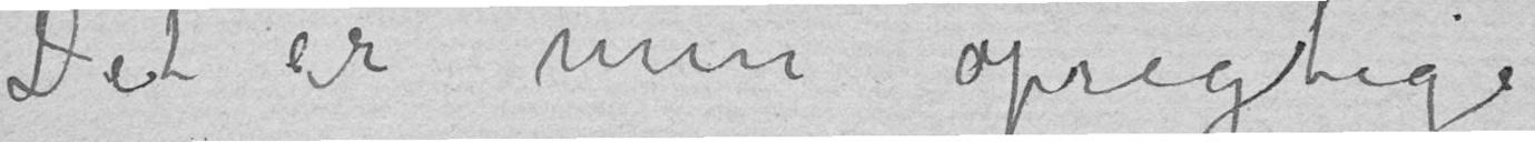Det er min oprigtige
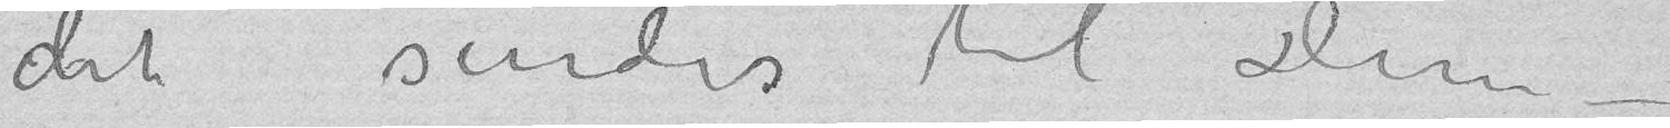det sendes til Dem –
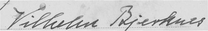Vilhelm Bjerknes
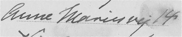Anne Maries vej 14
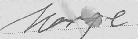Norge
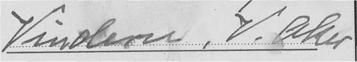Vindern, V. Aker
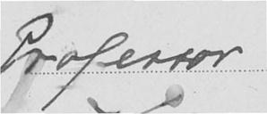Professor
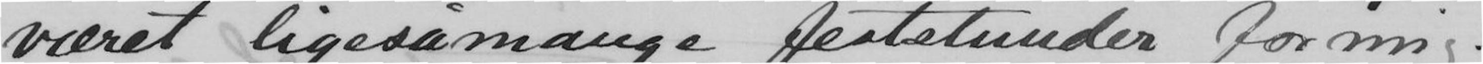været, ligesåmange feststunder for mig.
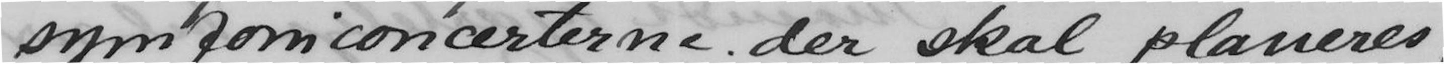symfoniconcerterne der skal planeres,
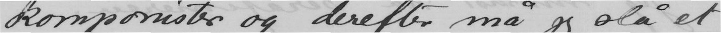komponister og derefter må jeg slå et
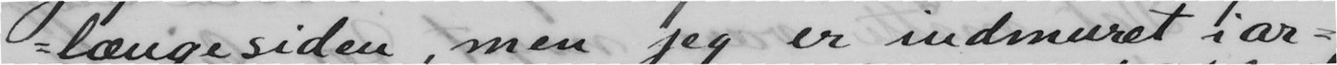længesiden, men jeg er indmuret i ar-
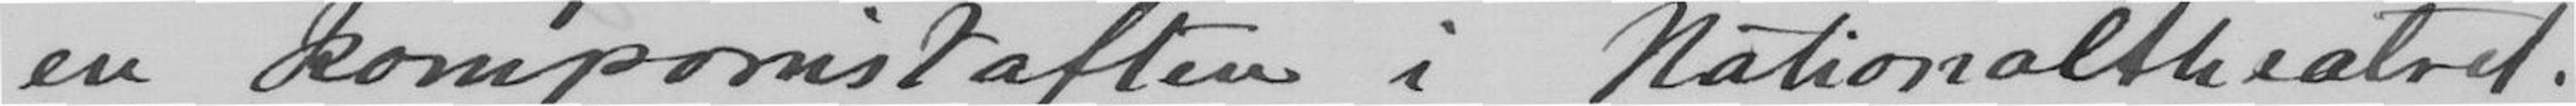en komponistaften i Nationaltheatret.
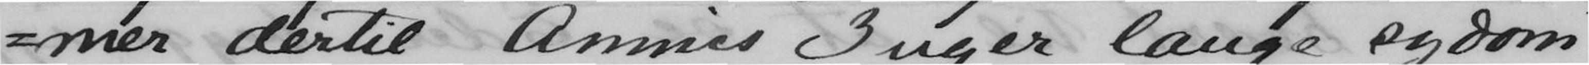mer dertil Annies 3 uger lange sygdom
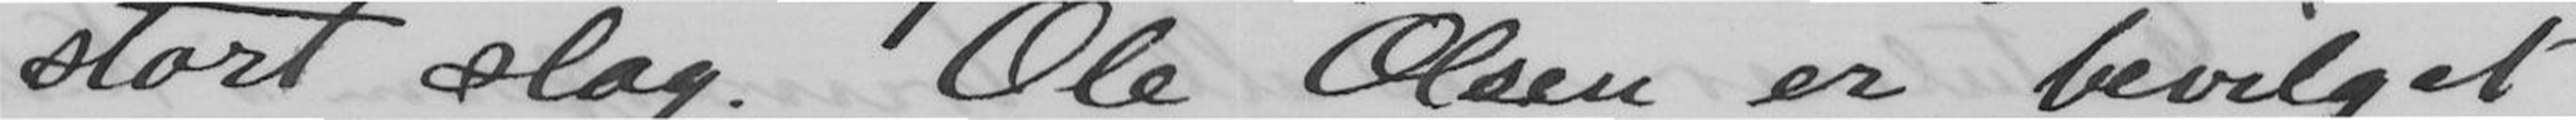stort slag. Ole Olsen er bevilget
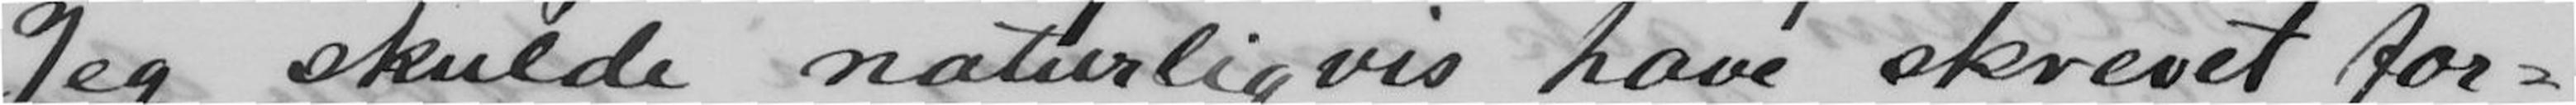Jeg skulde naturligvis have skrevet for-
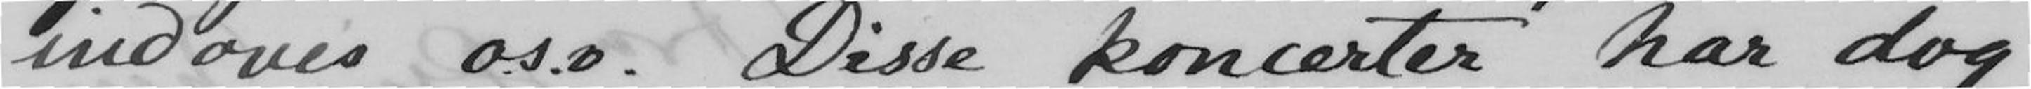indøves o.s.v. Disse koncerter har dog
